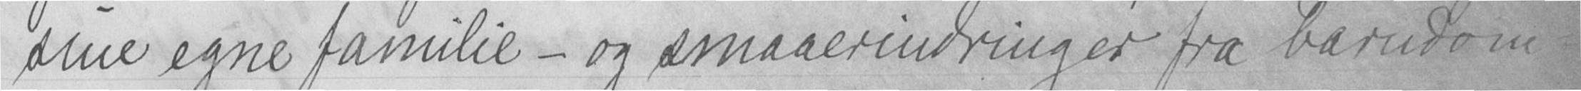sine egne familie - og smaaerindringer fra barndom-
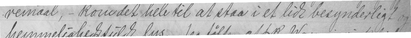remaal, - kom det hele til at staa i et lidt besynderligt og
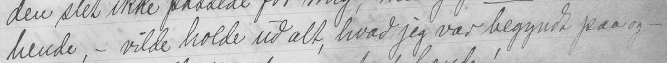hende, - vilde holde ud alt, hvad jeg var begyndt paa og -
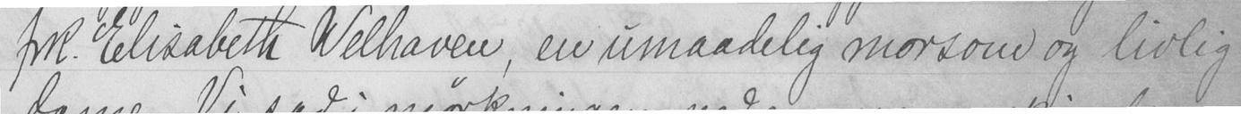frk. Elisabeth Welhaven, en umaadelig morsom og livlig
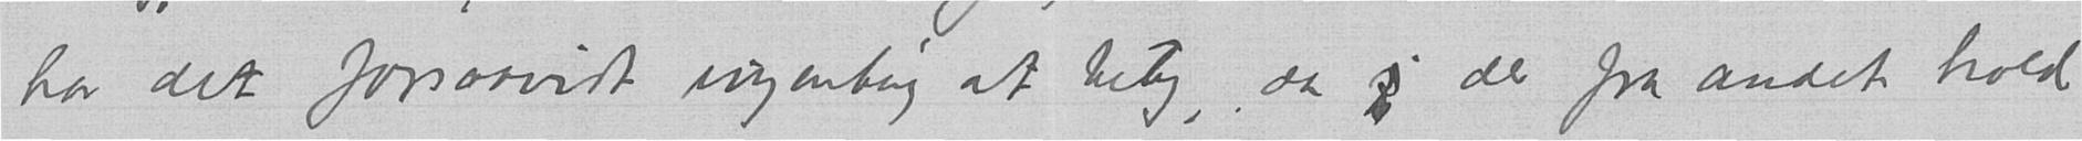har det forsaavidt ingenting at bety, da j der fra andet hold
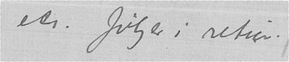etc. følger i retur.
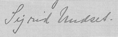Sigrid Undset.
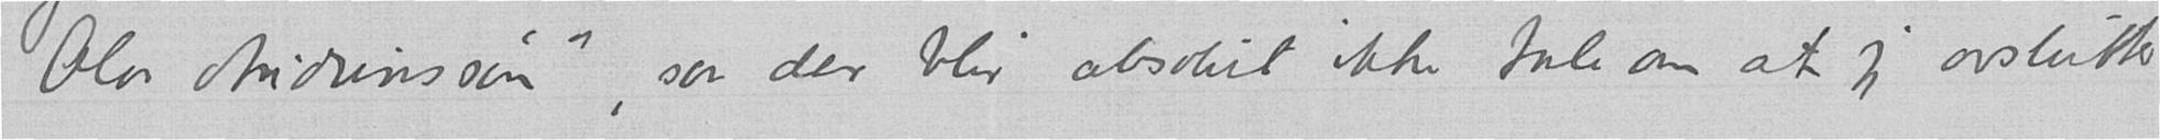"Olav Andunssøn», saa der blir absolut ikke tale om at jeg avslutter
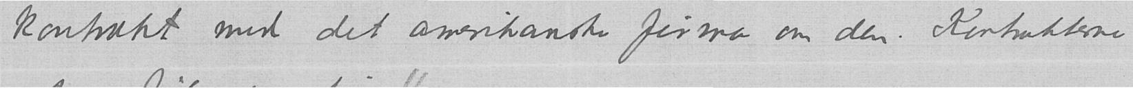kontrakt med det amerikanske firma om den. Kontrakterne
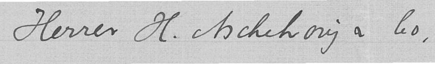Herrer H. Aschehoug & Co,
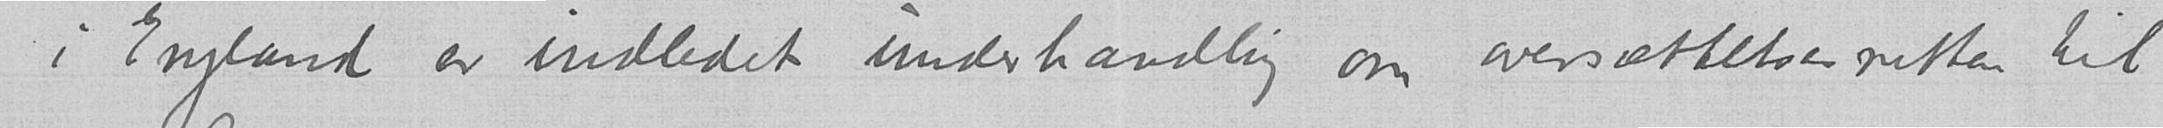i England er indledet underhandling om oversættelsesretten til
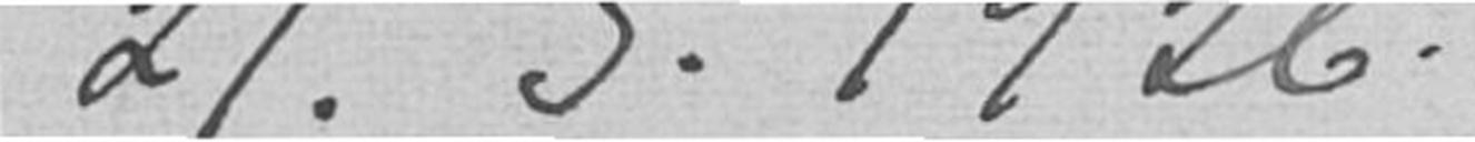21.3.1926.
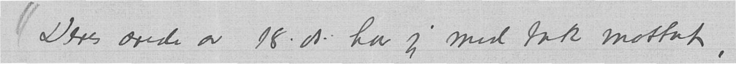Deres ærede av 18.ds. har jeg med tak mottat,
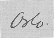Oslo.
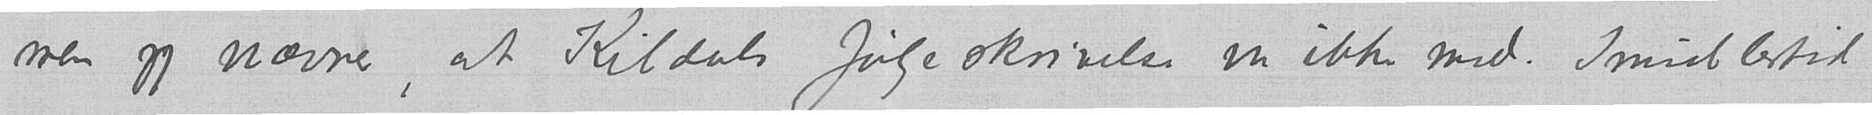men jeg nævner, at Kildals følgeskrivelse var ikke med. Imidlertid
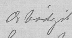Ærbødigst
